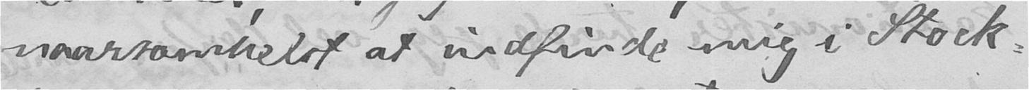naarsomhelst at indfinde mig i Stock-
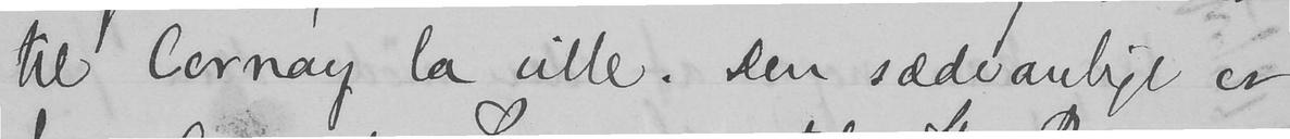til Cernay la ville. Den sædvanlige er
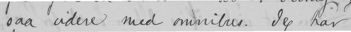saa videre med omnibus. Jeg har
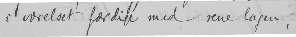i værelset færdige med rene lagen,
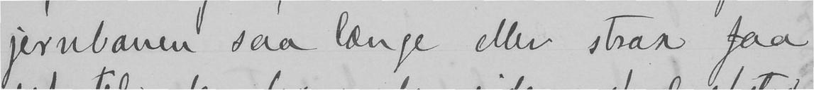jernbanen saa længe eller strax faa
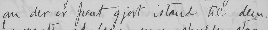om der er pent gjort istand til dem.
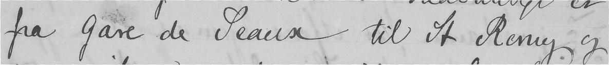fra Gare de Seaux til St Remy og
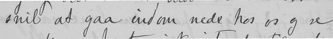snil at gaa indom nede hos os og se
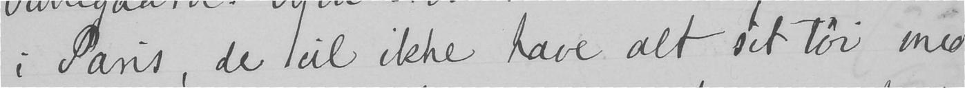i Paris, de vil ikke have alt sit tøi med
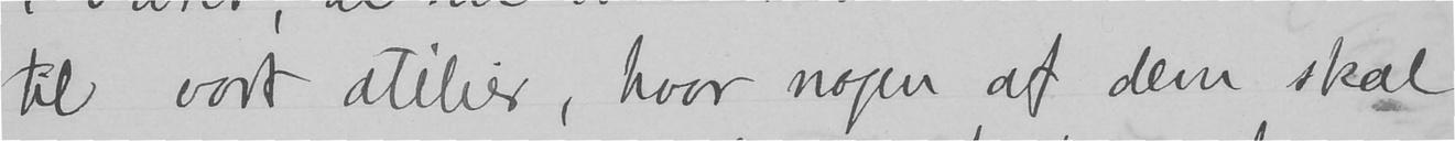til vort atélier, hvor nogen af dem skal
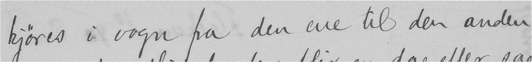kjøres i vogn fra den ene til den anden
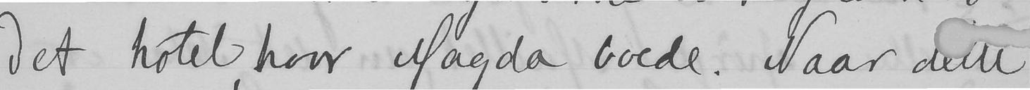det hotel hvor Magda boede. Naar dette
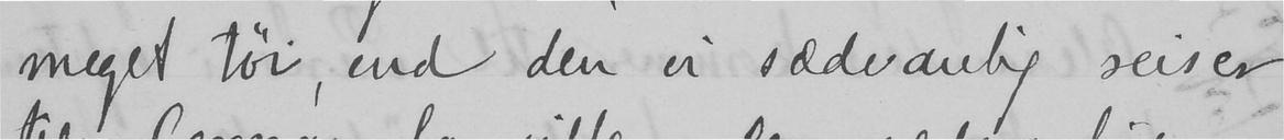meget tøi, end den vi sædvanlig reiser
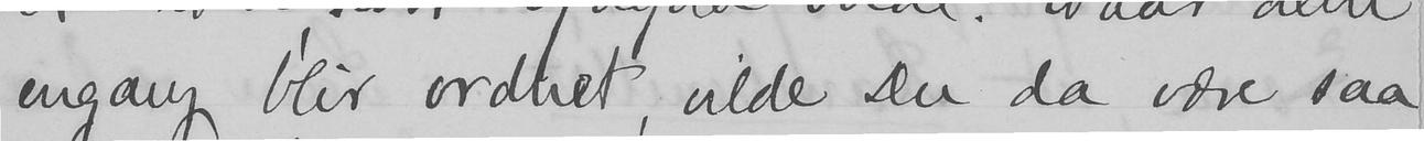engang blir ordnet, vilde Du da være saa
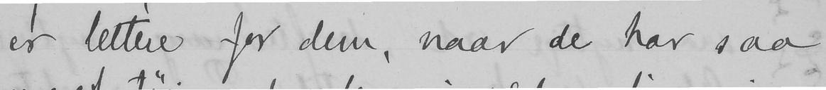er lettere for dem, naar de har saa
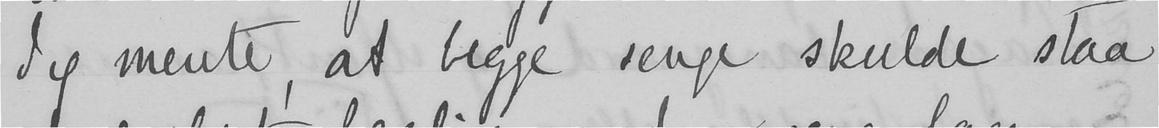Jeg mente, at begge senge skulde staa
